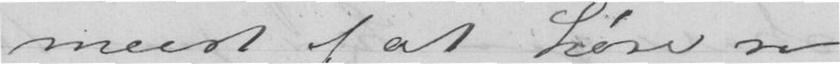meest af at høre sig
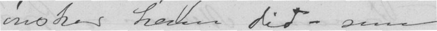ømsker ham did - men
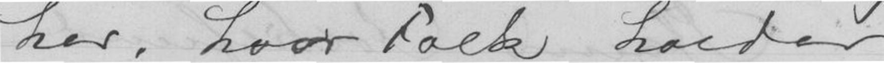her, hvor Folk holder
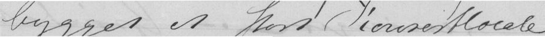bygget et stort Konsertlocale
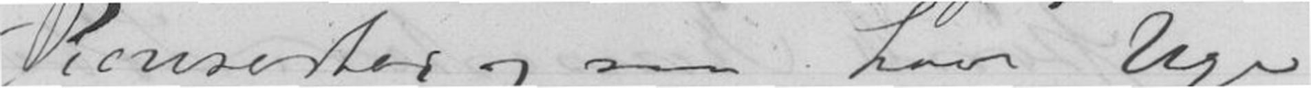Konserter og saa hver Uge
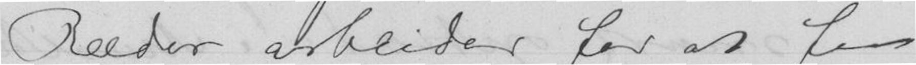Ræder arbeider for at faa
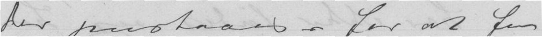der paastaaes - for at faa
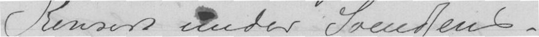Konsert under Svendsens
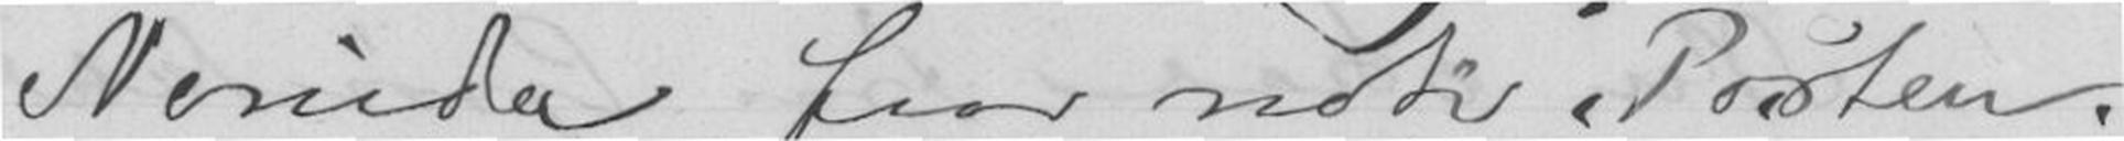Neruda faar nok Posten.
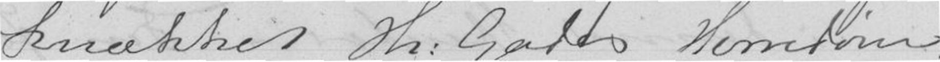knækket Hr: Gades Herredøm-
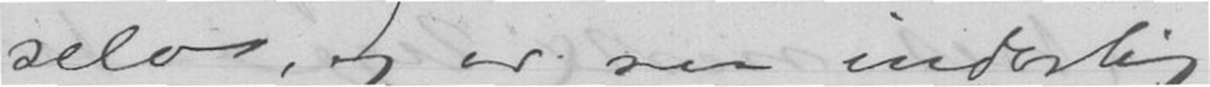selv, og er saa inderlig
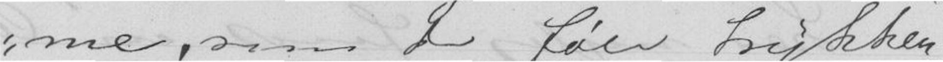me, som de føle trykken-
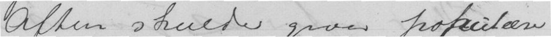Aften skulde give populære
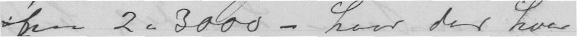paa 2=3000 - hvor der hver
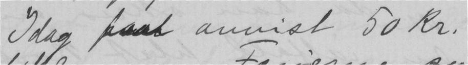Idag faat anvist 50 kr.
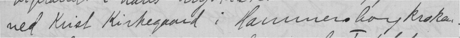ved Krist Kirkegaard i Hammersborgkroken.
